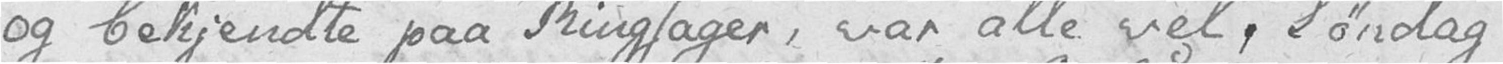og bekjendte paa Ringsager, var alle vel, Søndag
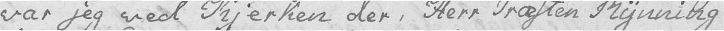var jeg ved Kjerken der, Herr Præsten Rynning
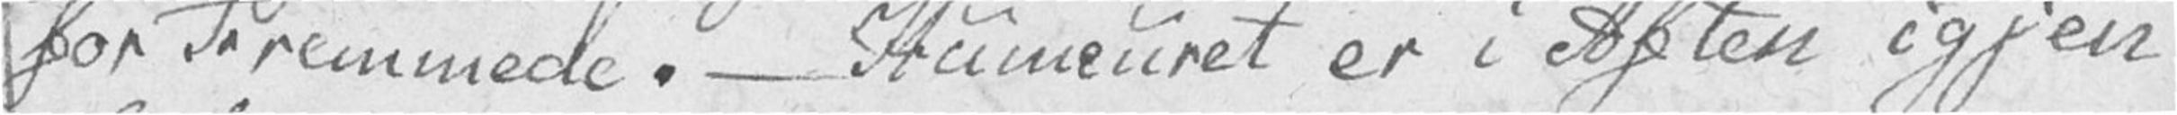for Fremmede. – Humeuret er i Aften igjen
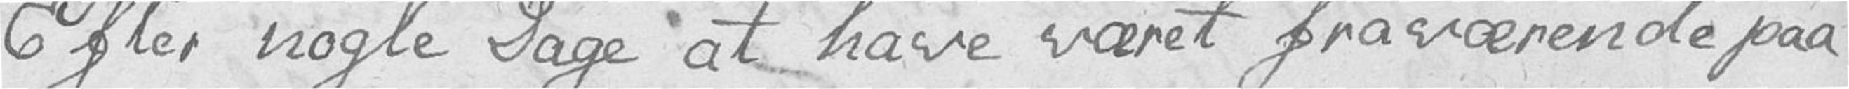Efter nogle Dage at have været fraværende paa
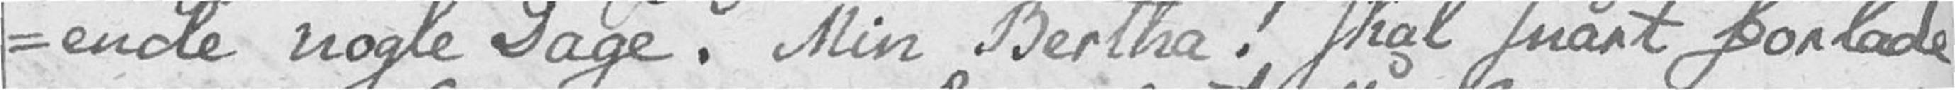-ende nogle Dage. Min Bertha! skal snart forlade
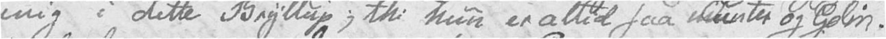mig i dette Bryllup, thi hun er altid saa munter og Golin.
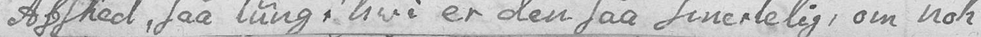Afsked, saa tung? hvi er den saa smertelig, om nok
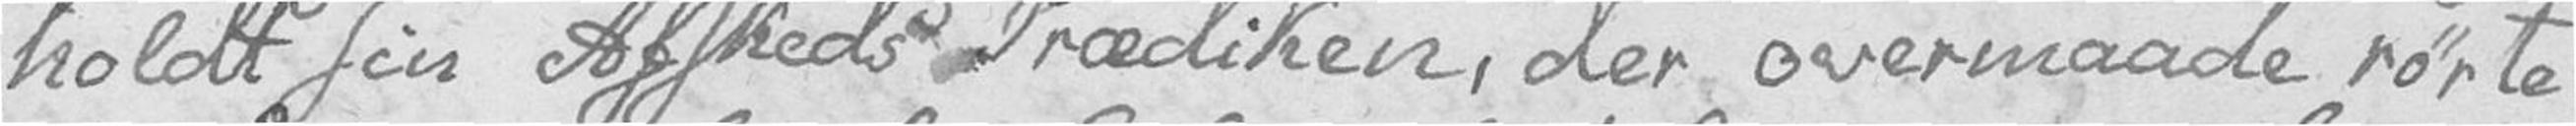holdt sin Afskeds Prædiken, der overmaade rørte
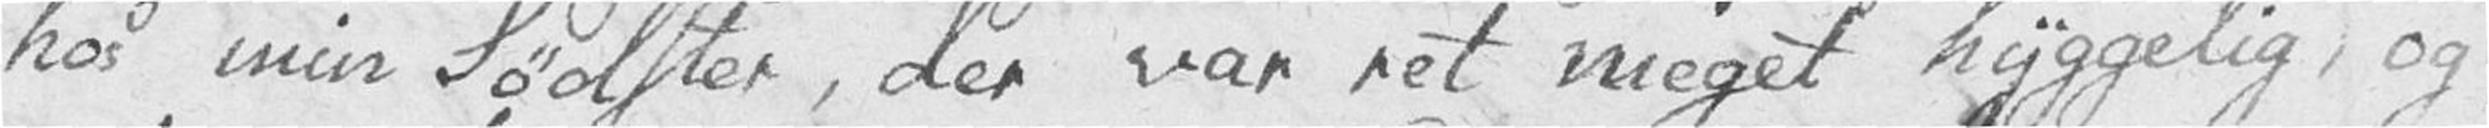hos min Sødster, der var ret meget hyggelig, og
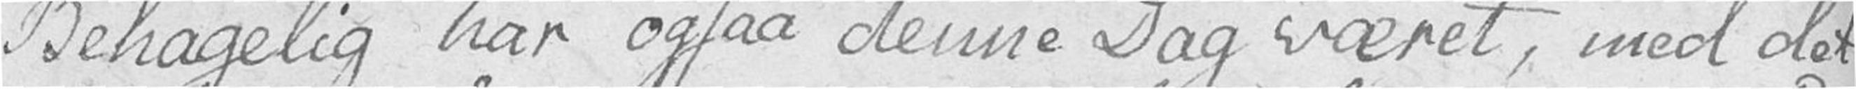Behagelig har ogsaa denne Dag været, med det
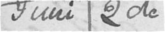Juni 2de
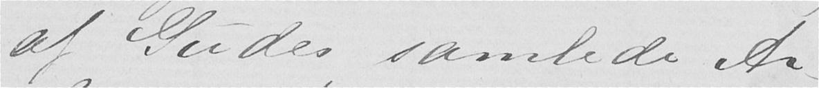af Gudes samlede Ar-
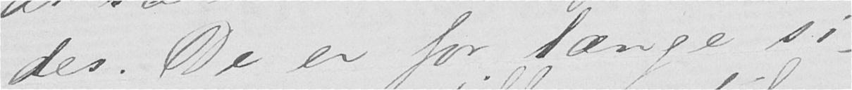des. De er for længe si-
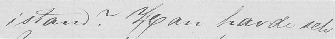istand? Han havde selv
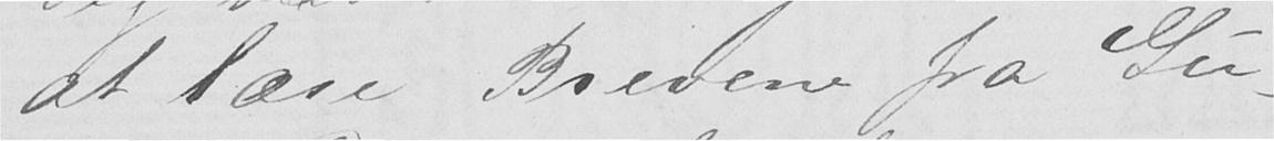at læse Brevene fra Gu-
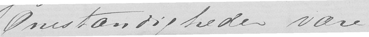Omstændigheder være
